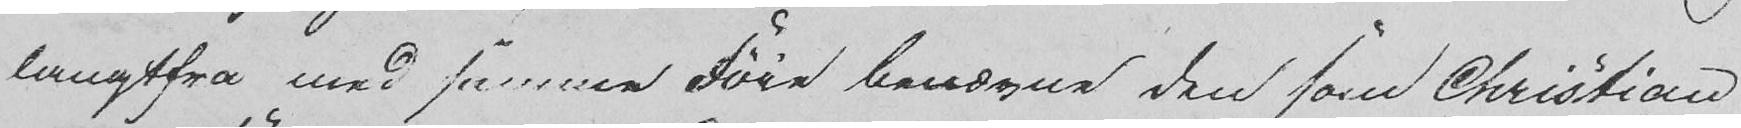langtfra med samme Føie benævne den som Christian
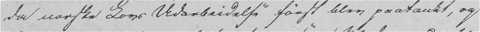da norske Lovs Udarbeidelse først blev paatænkt, og
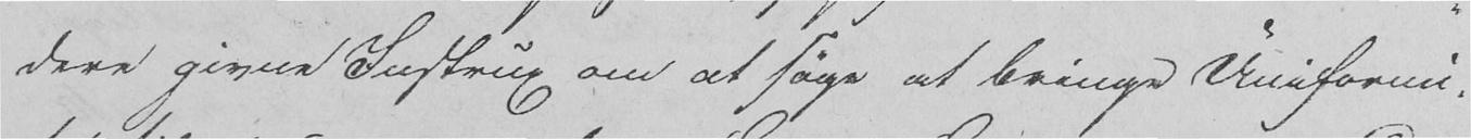dere givne Instrux om at søge at bringe Uniformi-
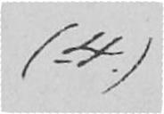(4.)
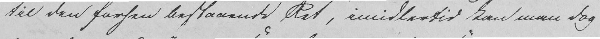til den forhen bestaaende Ret, imidlertid kan man dog
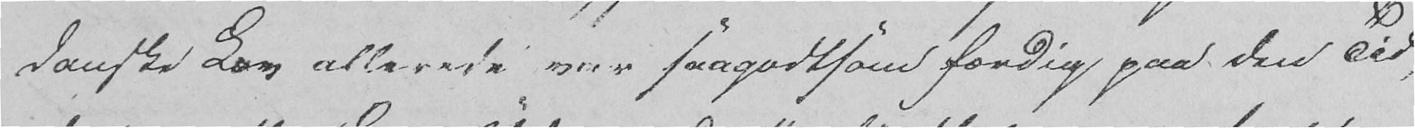danske Lov allerede var saagodtsom færdig paa den Tid
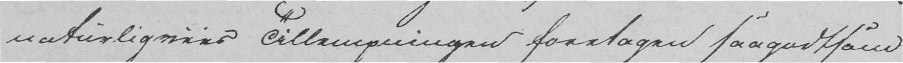naturligviis Tillempningen foretagen saagodtsom
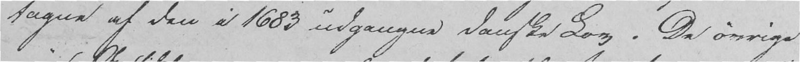tagne af den i 1683 udgangne danske Lov. De øvrige
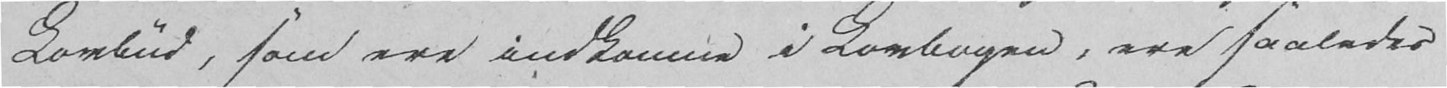Lovbud, som ere indkomne i Lovbogen, ere saaledes
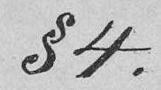§ 4.
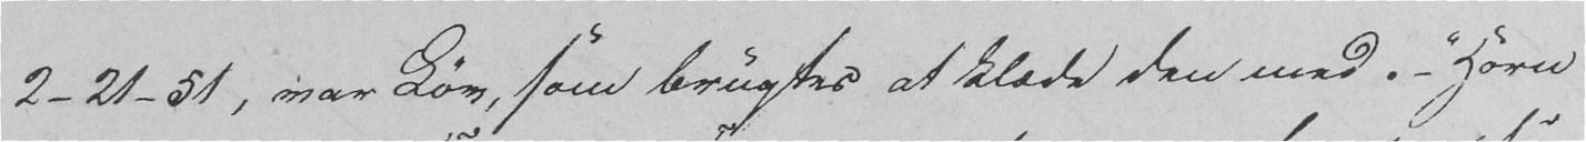2-21-51, var Lov, som brugtes at klæde den med. - "Horn
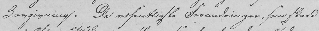Lovgivning. De væsentligste Forandringer, som skede
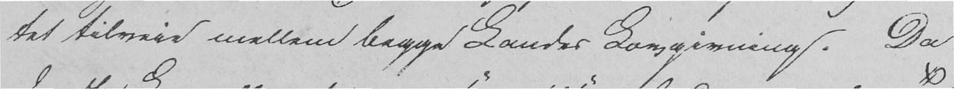tet tilveie mellem begge Landes Lovgivning. Da
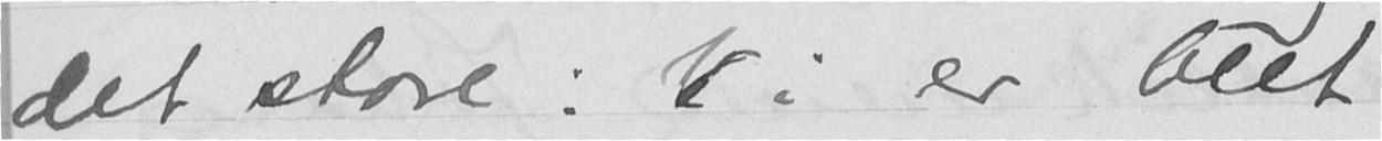det store: Vi er blit
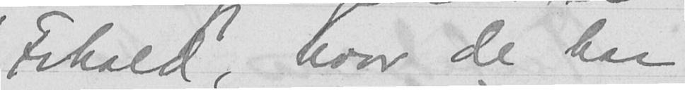Frhald, hvor de har
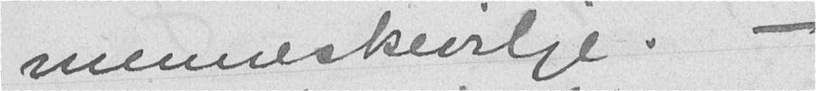menneskevilje. -
